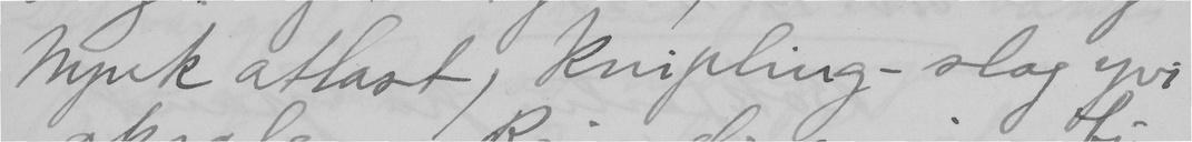Mjuk atlast, knipling-slag yvi
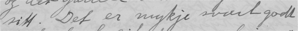sitt. Det er mykje svært godt
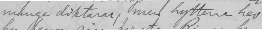mange diktarar, men hyttene hev
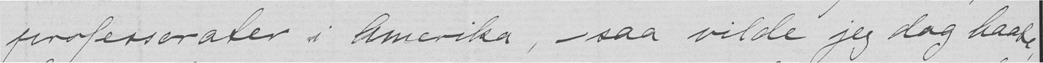professorater i Amerika, – saa vilde jeg dog haabe,
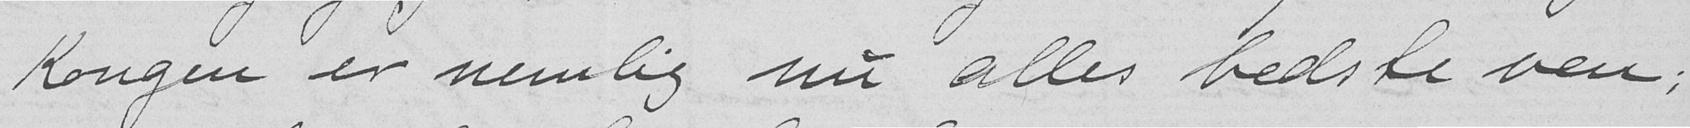Kongen er nemlig nu alles bedste ven;
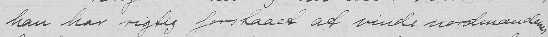han har rigtig forstaaet at vinde nordmændenes
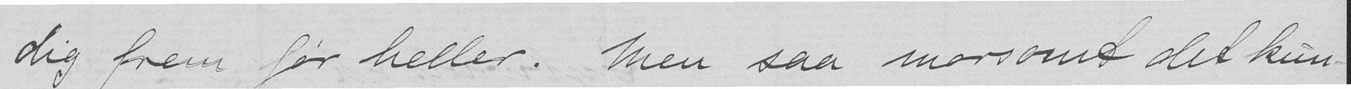dig frem før heller. Men saa morsomt det kun-
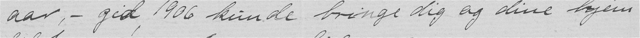aar, – gid 1906 kunde bringe dig og dine hjem
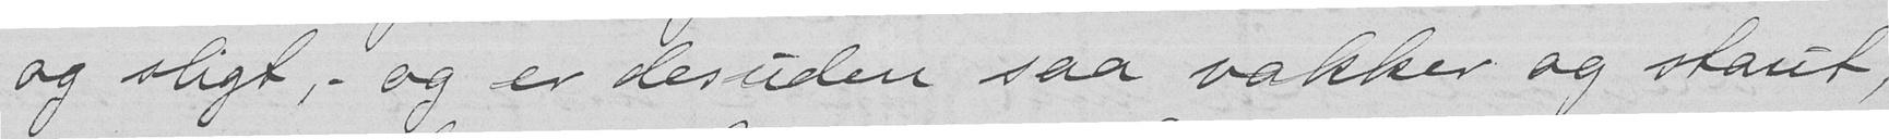og sligt, – og er desuden saa vakker og staut,
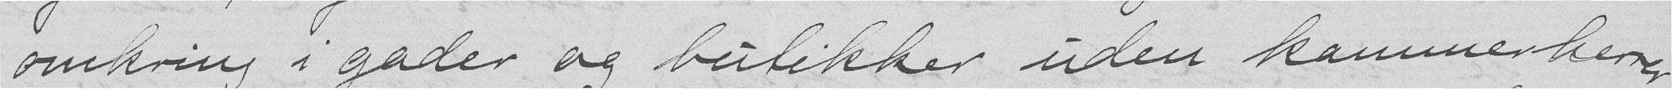omkring i gader og butikker uden kammerherrer
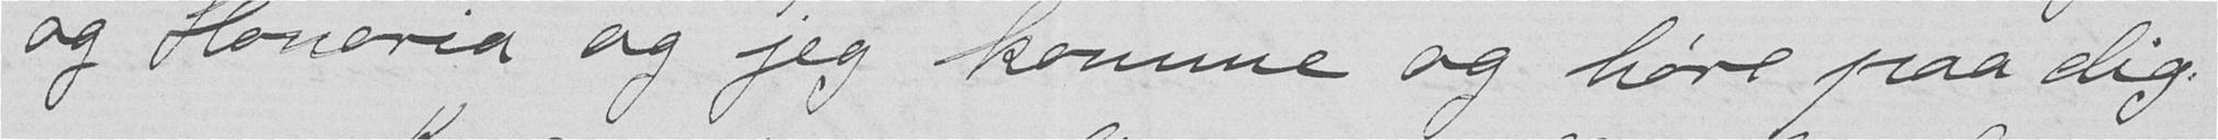og Honoria og jeg komme og høre paa dig.
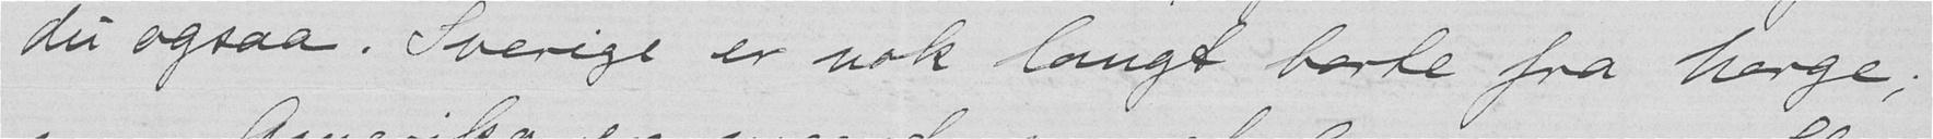du ogsaa. Sverige er nok langt borte fra Norge;
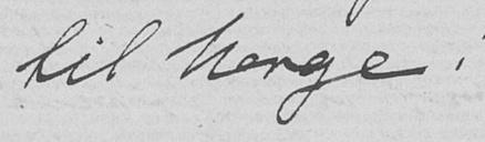til Norge!
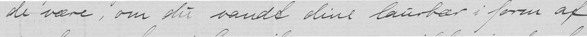de være, om du vandt dine laurbær i form af
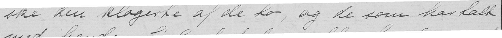ske den klogeste af de to, og de som har talt
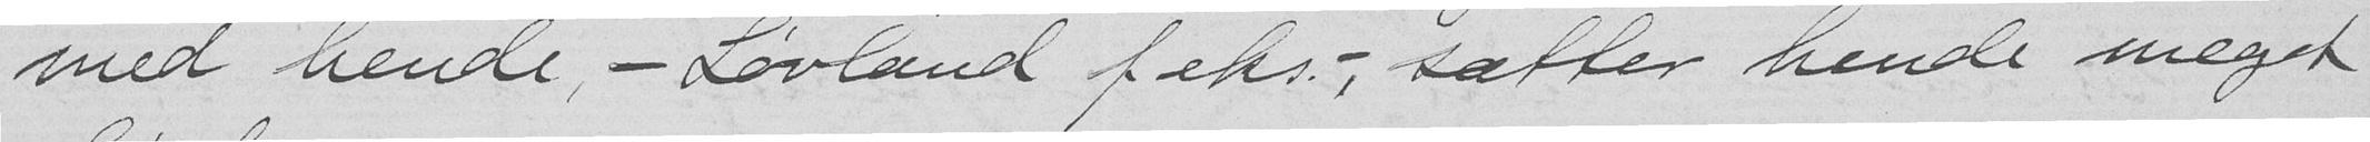med hende, – Løvland f eks. –, sætter hende meget
